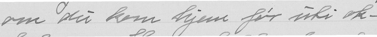om du kom hjem før uti ok-
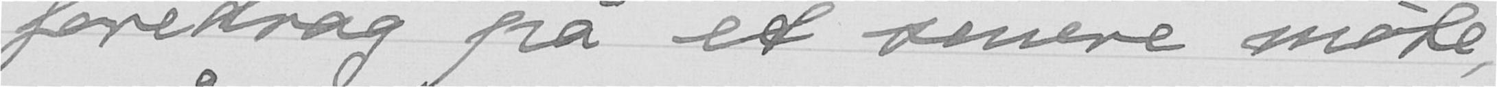foredrag på et senere møte,
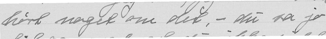hørt noget om det, - du sa jo
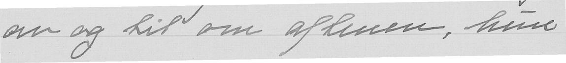av og til om aftenen, hun
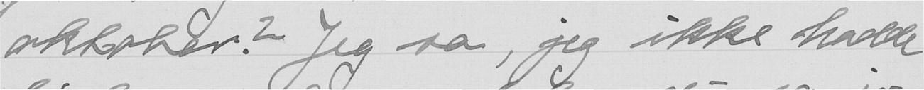oktober? Jeg sa, jeg ikke hadde
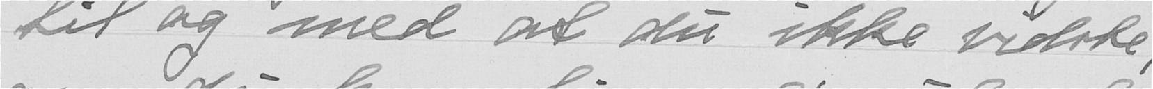til og med at du ikke vidste,
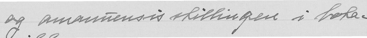og amanuensisstillingen i bota-
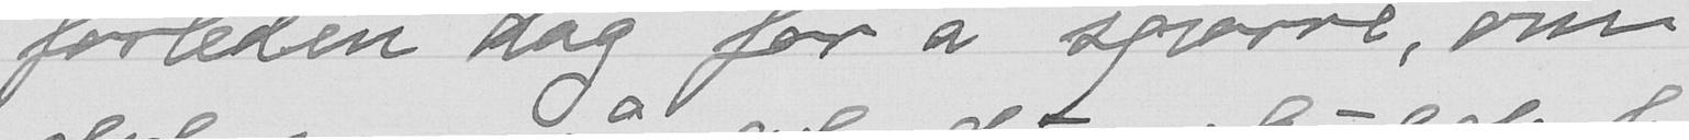forleden dag for å spørre, om
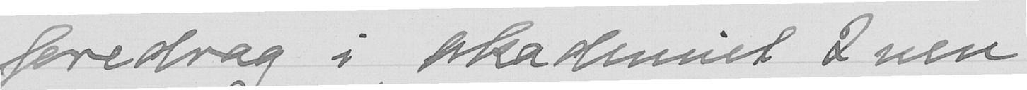foredrag i Akademiet 2nen
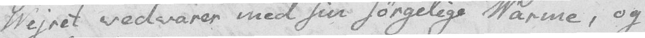Vejret vedvarer med sin sørgelige Varme, og
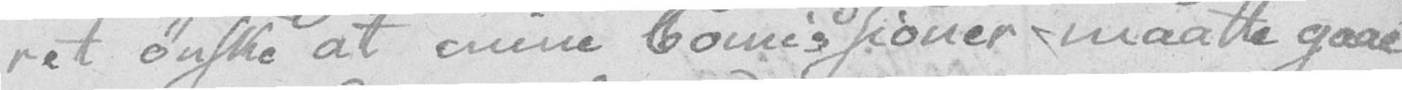ret ønske at mine Comissioner maatte gaae
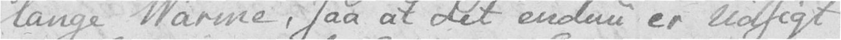lange Varme, saa at det endnu er Udsigt
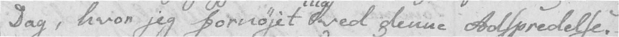Dag, hvor jeg fornøjet mig ved denne Adspredelse.
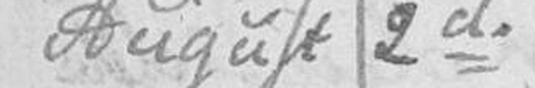August 2de
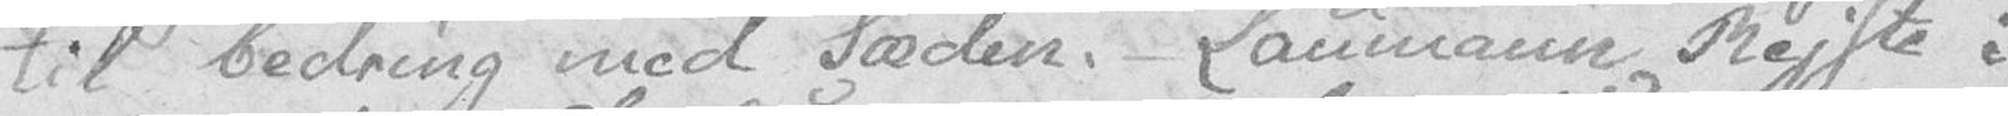til bedring med Sæden. Laumann Rejste i
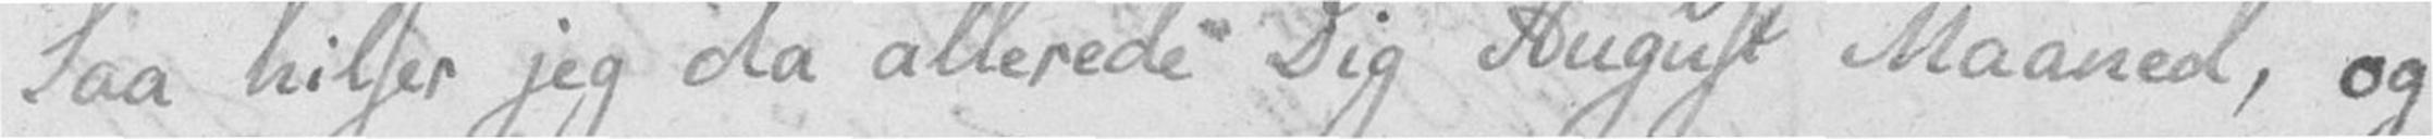Saa hilser jeg da allerede Dig August Maaned, og
